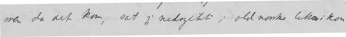men da det kom, sat jeg nedsyltet i oldnorske leksikon
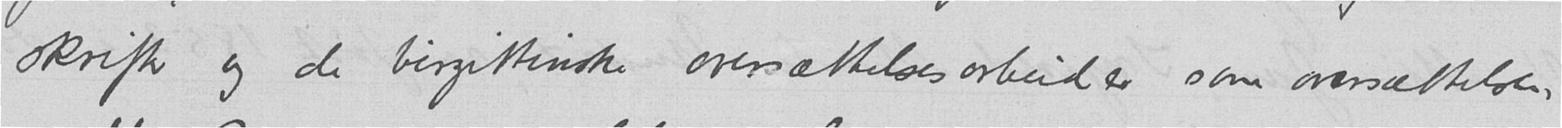skrifter og de birgittinske oversættelsesarbeider som oversættelsen
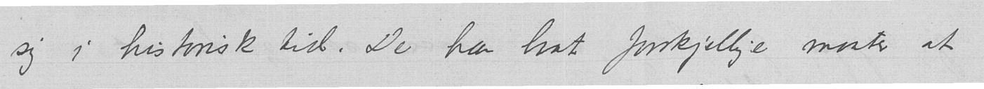sig i historisk tid. De har hat forskjellige maater at
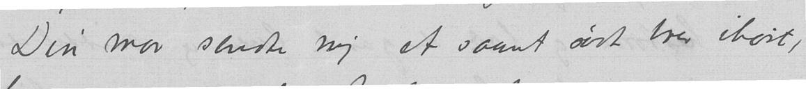Din mor sendte mig et saant sødt brev ihøst,
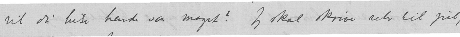vil du hilse hende saa meget? Jeg skal skrive selv til jul,
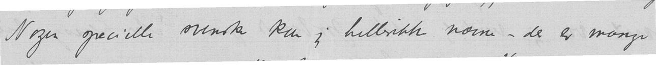Nogen specielle svenske kan jeg hellerikke nevne - der er mange
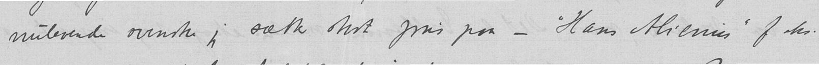nulevende svenske jeg sætter stor pris paa - "Hans Alienus" f eks.
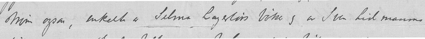strøm ogsaa, enkelte av Selma Lagerløvs bøker og av Sven Lidmanns
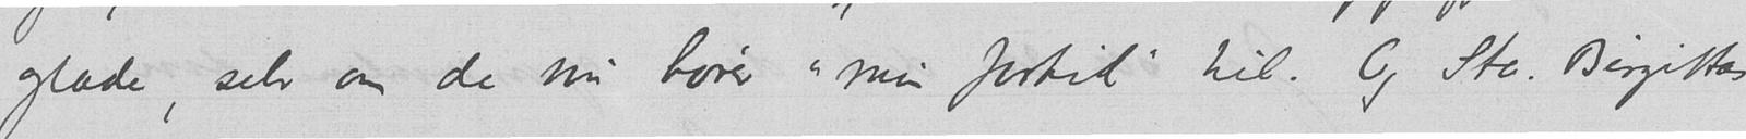glæde, selv om de nu hører "min fortid" til. Og Sta. Birgittas
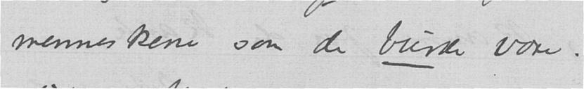menneskene som de burde være.
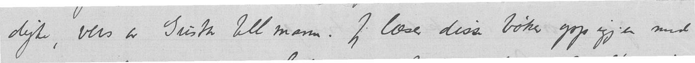digte, vers av Gustav Ullmann. Jeg læser disse bøker opp igjen med
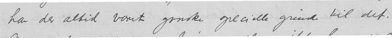har der altid været ganske specielle grunde til det.
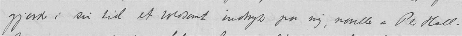gjorde i sin tid et voldsomt indtryk paa mig, noveller av Per Hall-
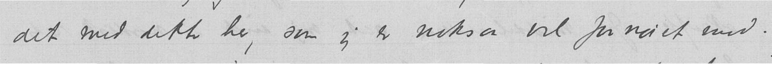det med dette her, som jeg er noksaa vel fornøiet med.
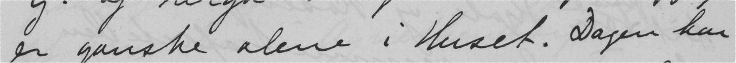er ganske alene i Huset. Dagen har
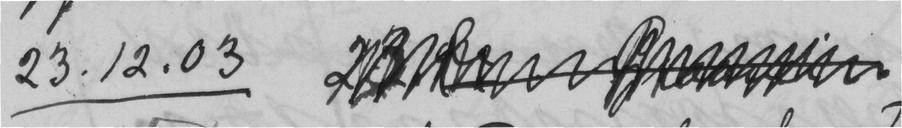23.12.03
